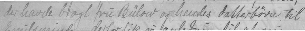der havde bragt fru Bulow og hendes datterbørn til
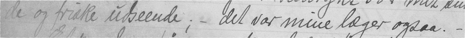de og friske udseende; - det var mine læger ogsaa. -
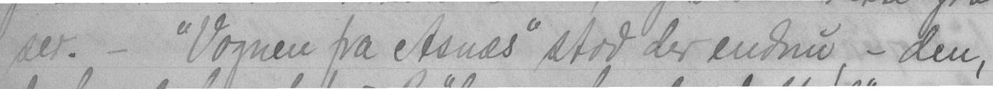ser. - "Vognen fra Asnæs" stod der endnu, - den,
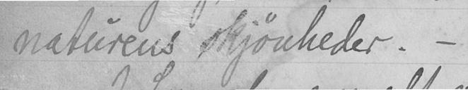naturens skjønheder. -
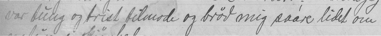var tung og trist tilmode og brød mig saare lidet om
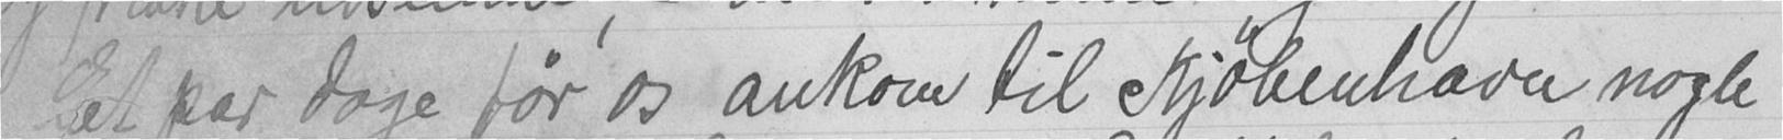et par dage før os ankom til Kjøbenhavn nogle
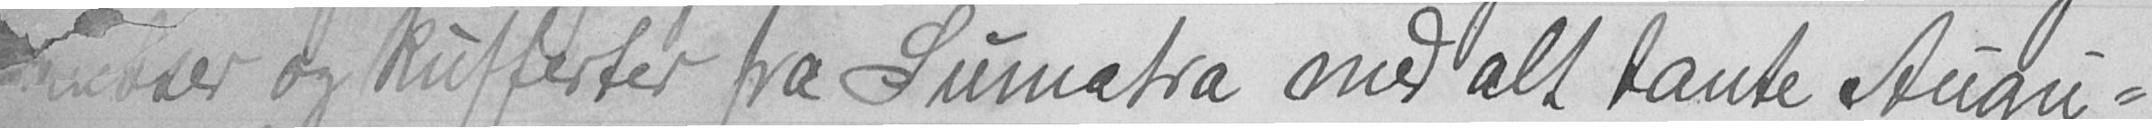kudser og kufferter fra Sumatra med alt tante Augu-
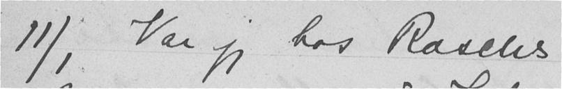11/1 Var jeg hos Raschs
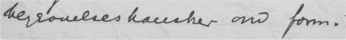begravelseshansker om form.
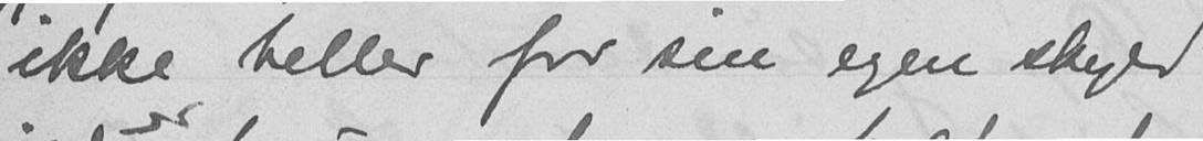ikke heller for sin egen skyld
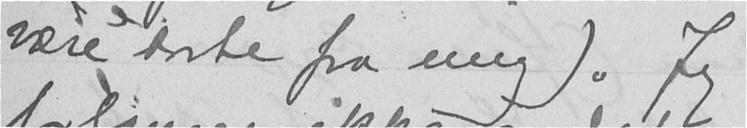være borte fra mig) "Jeg
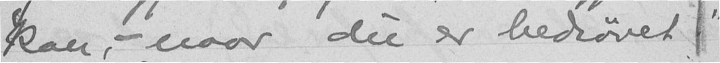kan, - naar du er bedrøvet."
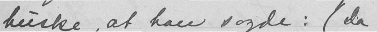huske, at han sagde: (da
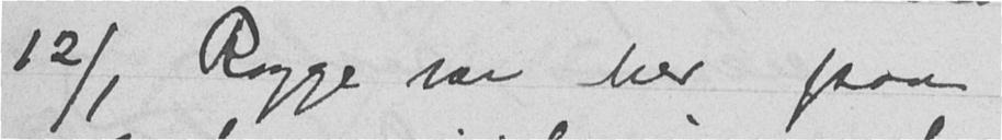12/1 Rogge var her paa
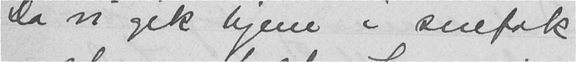Da vi gik hjem i snefok
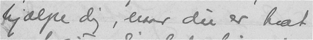hjælpe dig, naar du er træt
